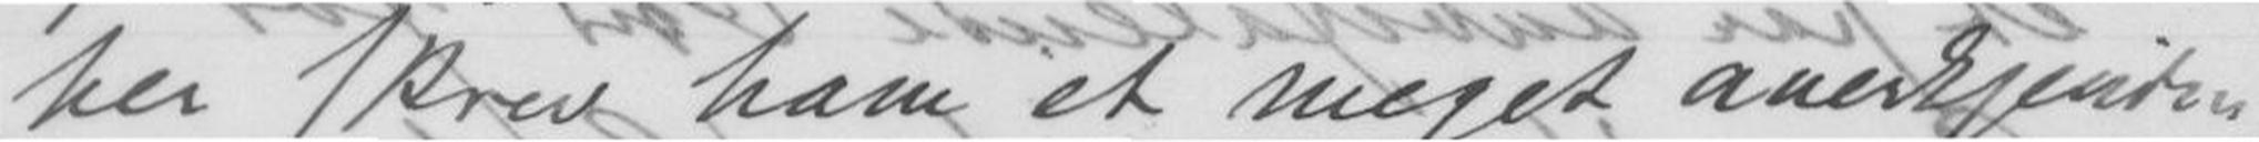her Skrev ham et meget anerkjenden-
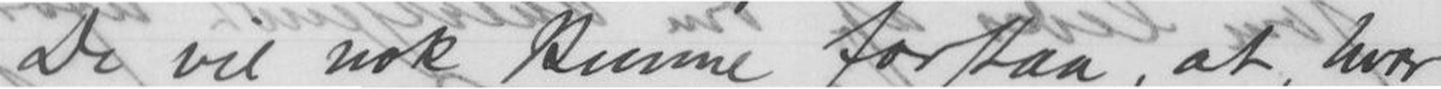De vil nok kunne forstaa, at hvor
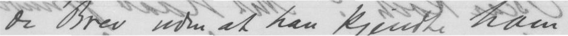de Brev om at han kjendte ham
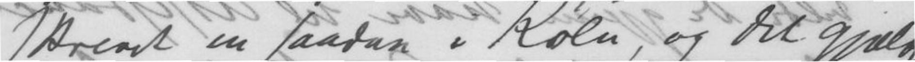skrevet en saadan i Köln, og det gjælder
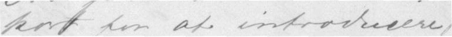kort for at introdusere
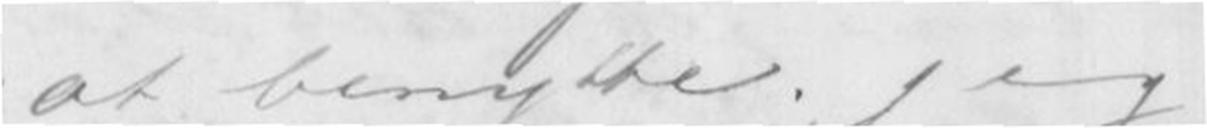at benytte; jeg
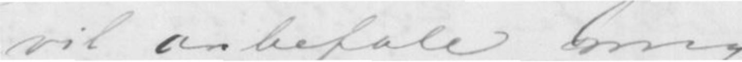vil anbefale mig
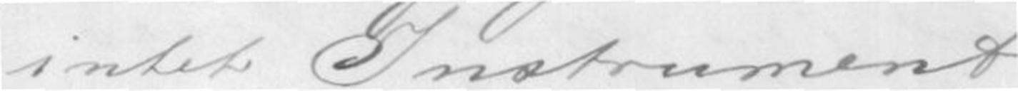intet Instrument
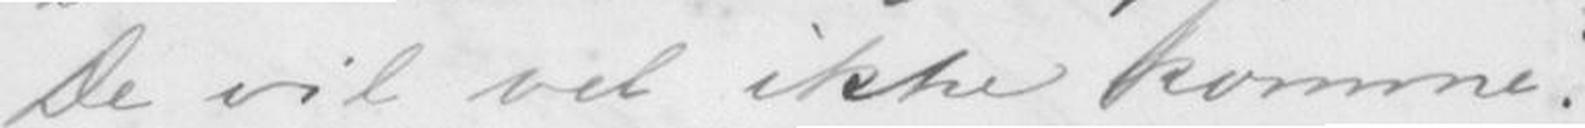De vil vel ikke komme?
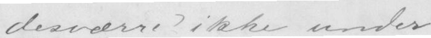desværre ikke under
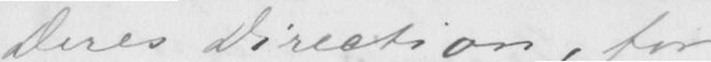Deres Direction, for
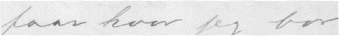faar hvor jeg bor.
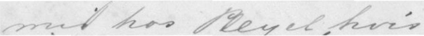mig hos Pleyel, hvis
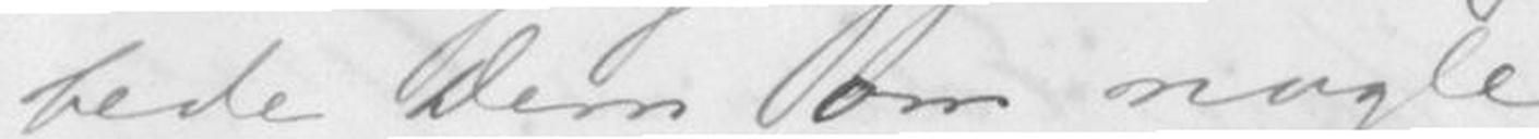bede Dem om nogle
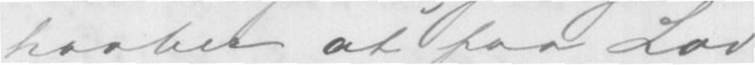haaber at faa Lov
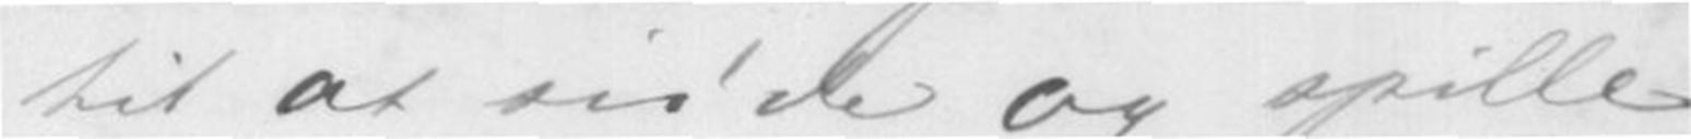til at sidde og spille
